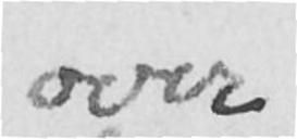over
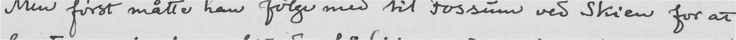Men først måtte han følge med til Fossum ved Skien for at
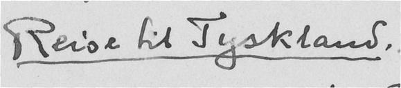Reise til Tyskland.
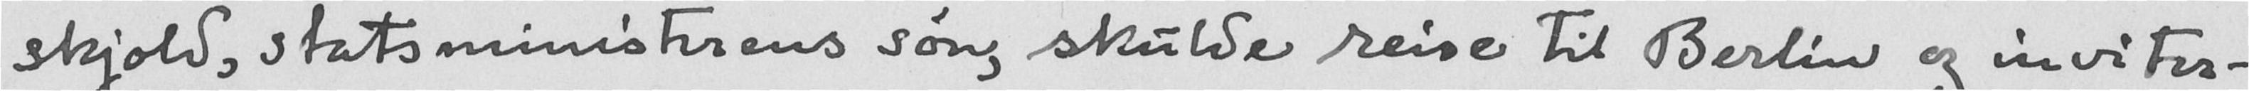skjold, statsministerens søn, skulde reise til Berlin og inviter-
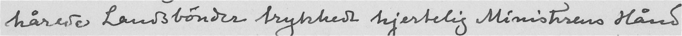hårede Landsbønder trykkede hjertelig Ministerens Hånd
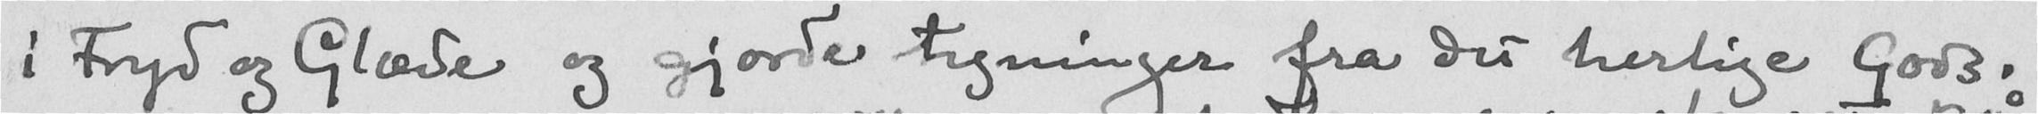i Fryd og Glæde og gjorde tegninger fra det herlige Gods.
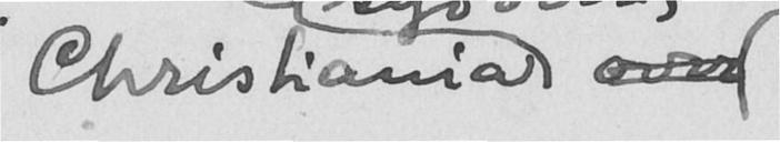Christiania over
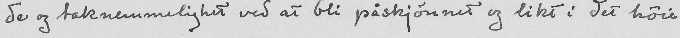de og taknemmelighet ved at bli påskjønnet og likt i det høie
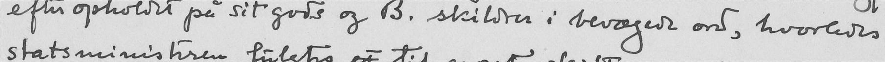efter opholdet på sit gods og B. skildrer i bevægede ord, hvorledes
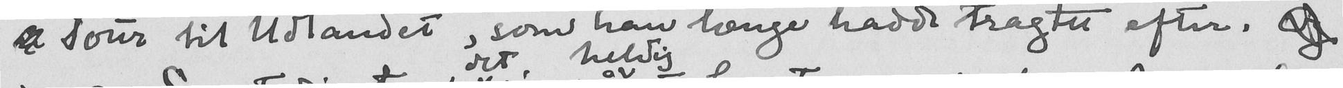Tour til Udlandet, som han længe hadde tragtet efter. Og
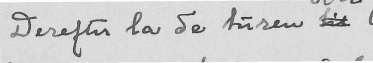Derefter la de turen til
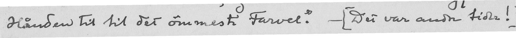Hånden til til det ømmeste Farvel." - (Det var andre tider!)
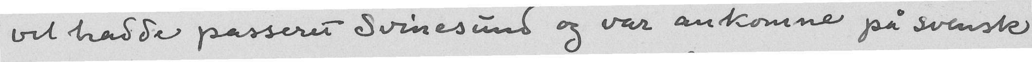vel hadde passeret Svinesund og var ankomne på svensk
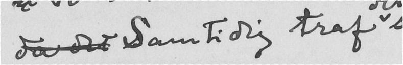da det Samtidig traf
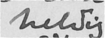heldig
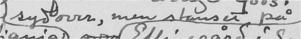sydover, men stanset på
